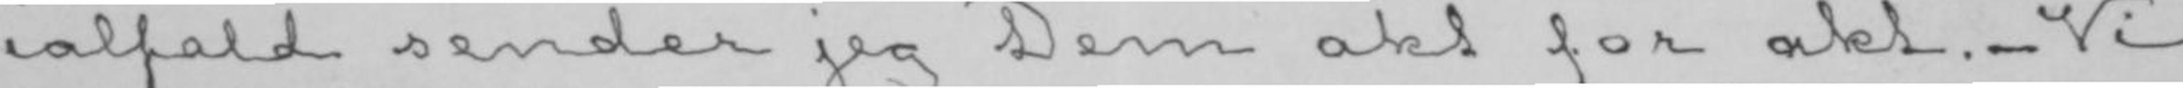ialfald sender jeg Dem akt for akt.- Vi
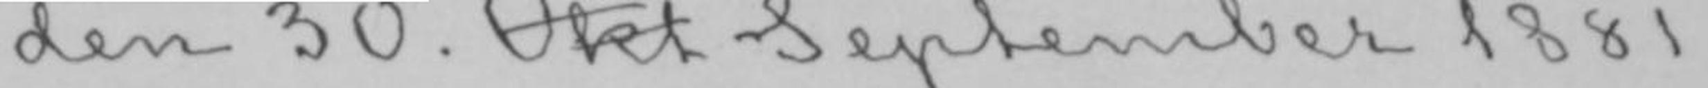den 30. Okt September 1881.
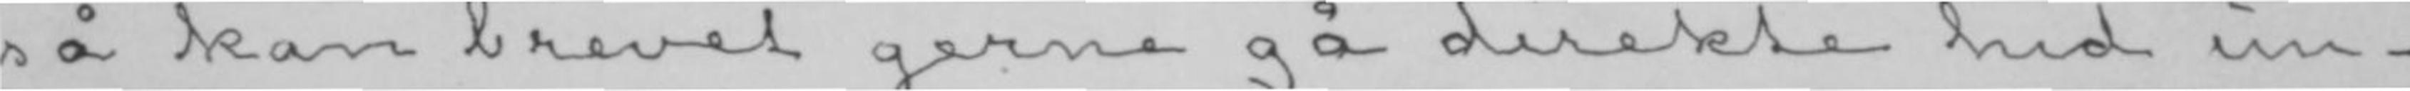så kan brevet gerne gå direkte hid un-
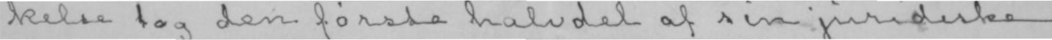kelse tog den første halvdel af sin juridiske
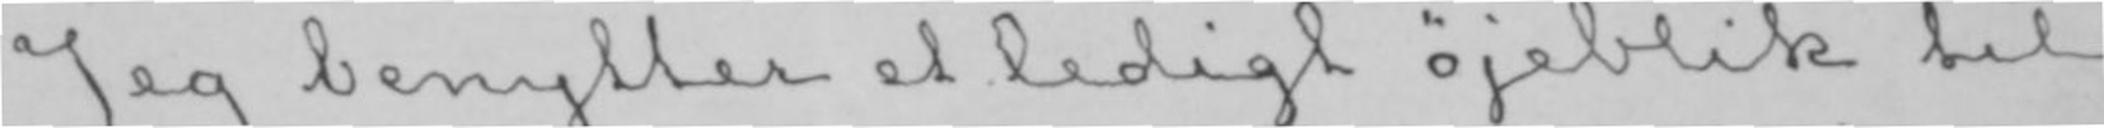Jeg benytter et ledigt øjeblik til
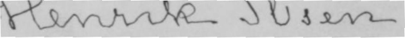Henrik Ibsen.
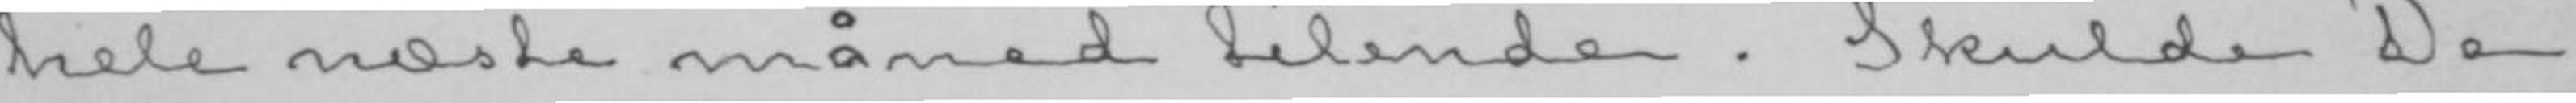hele næste måned tilende. Skulde De
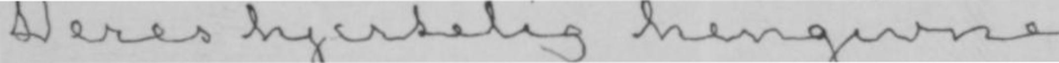Deres hjertelig hengivne
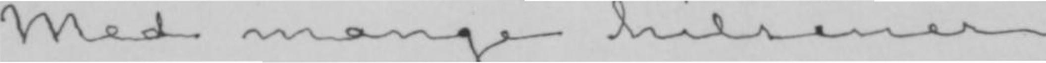Med mange hilsener
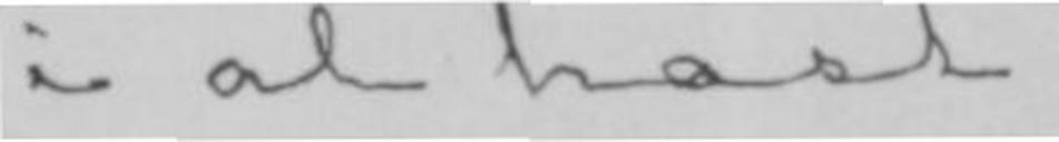i al hast,
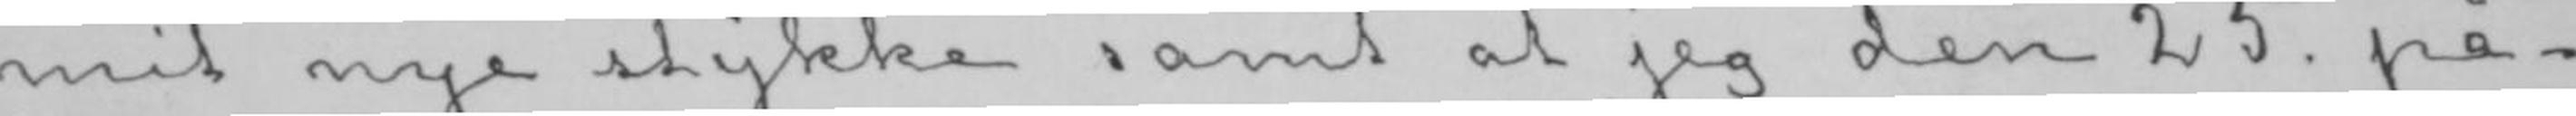mit nye stykke samt at jeg den 25. på-
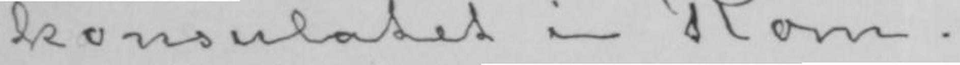konsulatet i Rom.
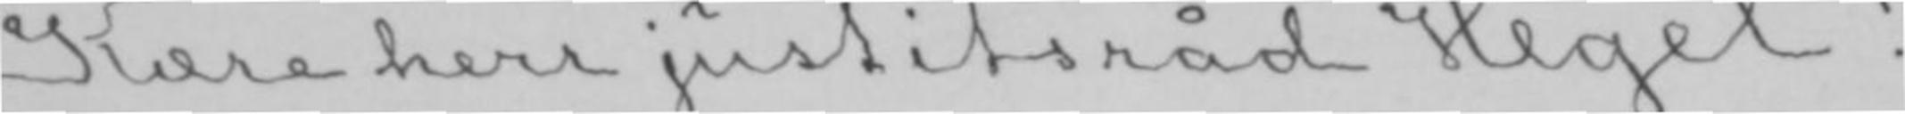Kære herr justitsråd Hegel!
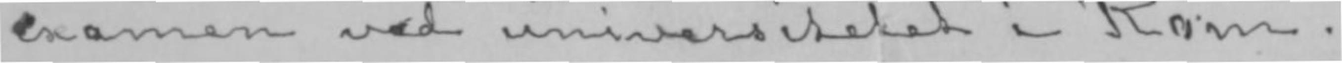examen ved universitetet i Rom.
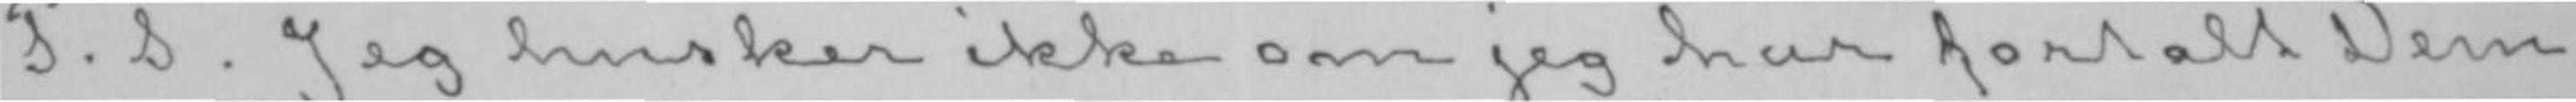P. S. Jeg husker ikke om jeg har fortalt Dem
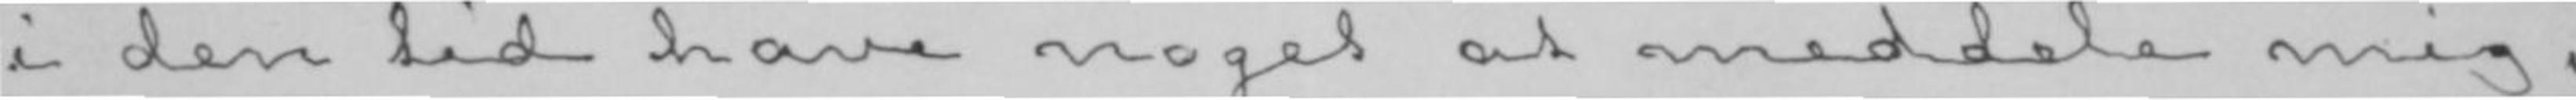i den tid have noget at meddele mig,
